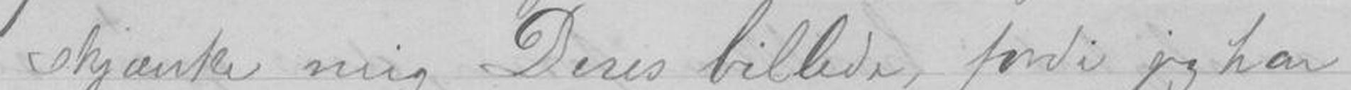skjænke mig Deres billede, fordi jeg har
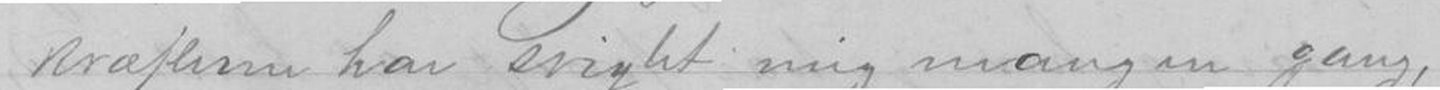kræfterne har svigtet mig mangen gang,
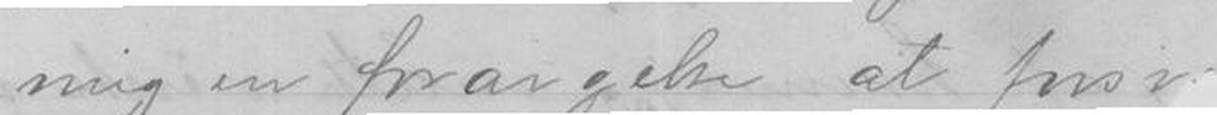mig en forargelse at fors
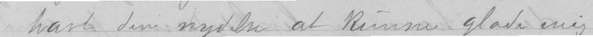havt den nydelse at kunne glæde mig
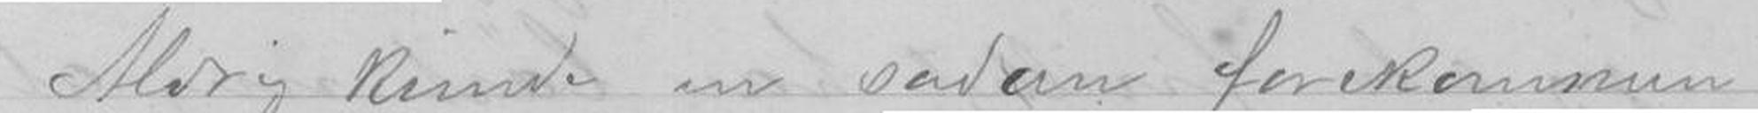Aldrig kunde en sådan forekommen
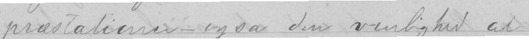præstationer - og så den venlighed at
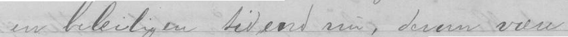en beleiligen tid end nu, derom være
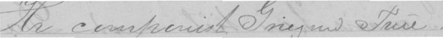Hr Componist Grieg med Frue
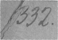332.
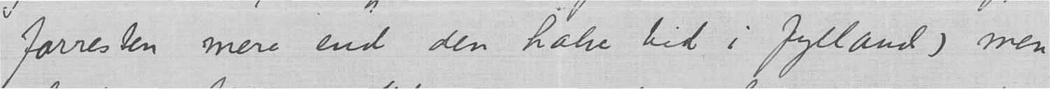forresten mere end den halve tid i Jylland) men
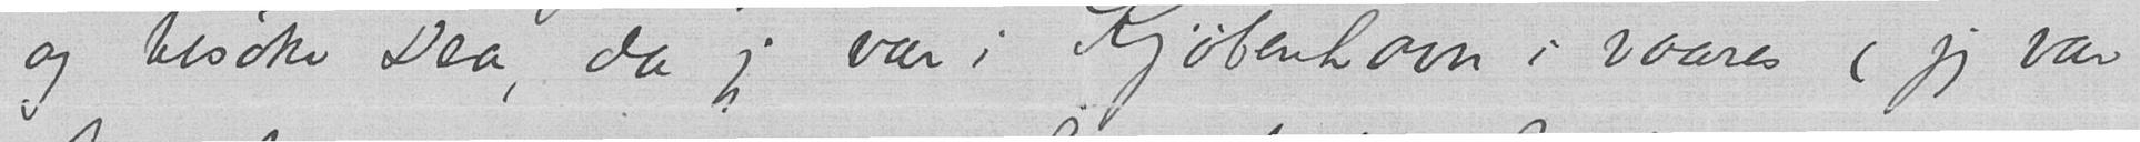og besøke Dea, da jeg var i Kjøbenhavn i vaares (jeg var
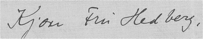Kjære Fru Hedberg,
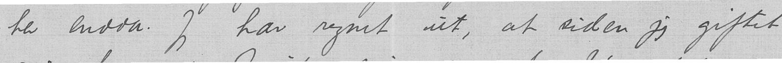her endda. Jeg har regnet ut, at siden jeg giftet
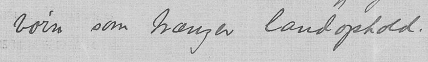børn, som trænger landophold.
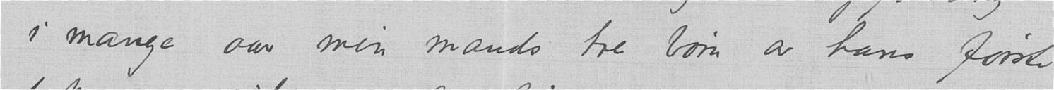- i mange aar min mands tre børn av hans første
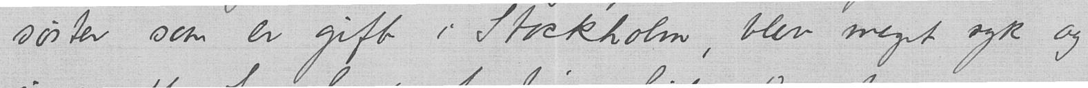søster som er gift i Stockholm blev meget syk og
